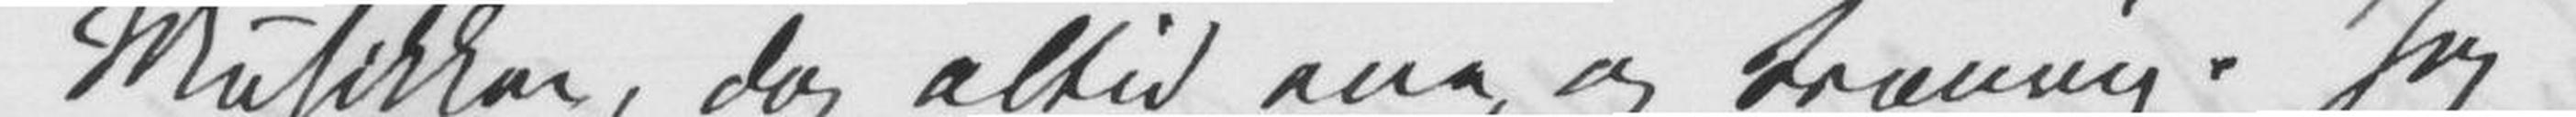Musikken, dog altid ene og traurig. Jeg
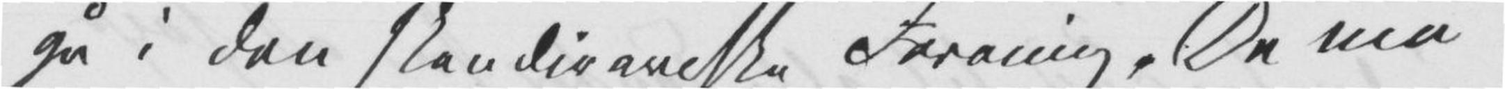gå i den skandinaviske Forening. De må
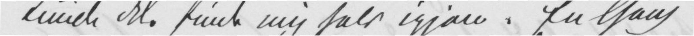kunde ikke finde mig selv igjen. En Gang
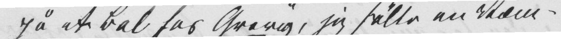på et Bal hos Grevy, jeg følte en Væm¬
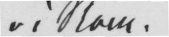i Rom
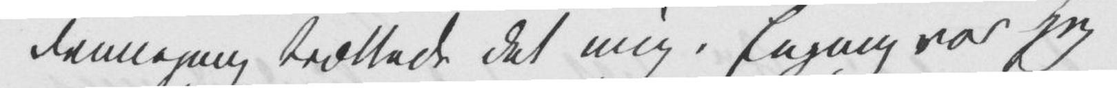dennegang trættede mig. Engang var jeg
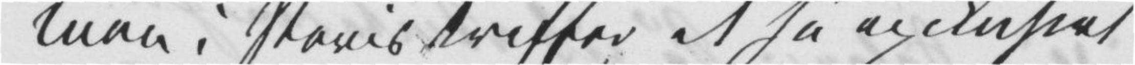man i Paris treffer et så exclusivt
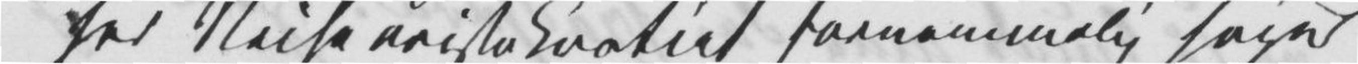her Reisearistokratiet fornemmelig søger
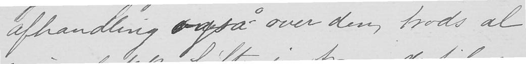afhandling også over den, trods al
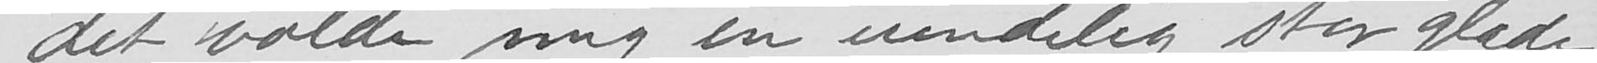det volde mig en uendig stor glede
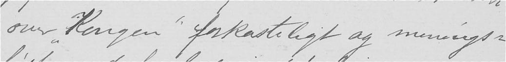over "Kongen" forkasteligt og menings-
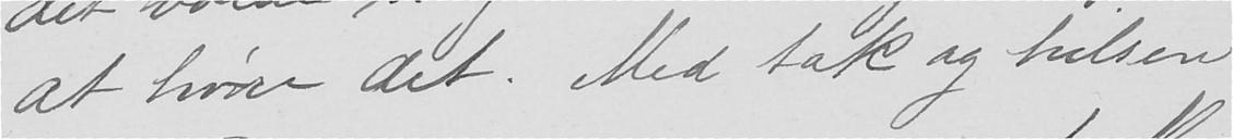at høre det. Med tak og hilsen. d
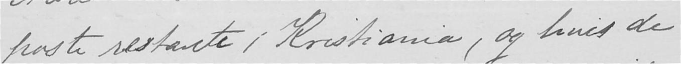poste restante i Kristiania, og hvis de
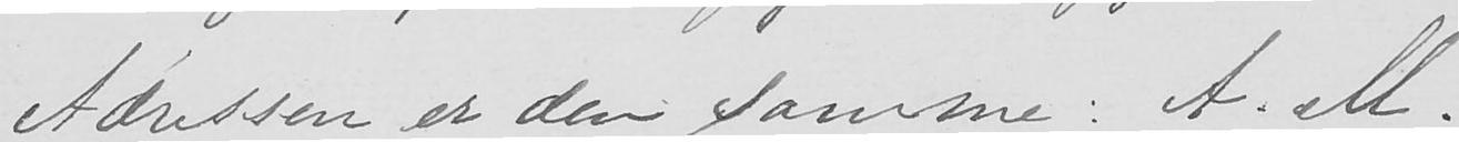Adressen er den samme: A. M.
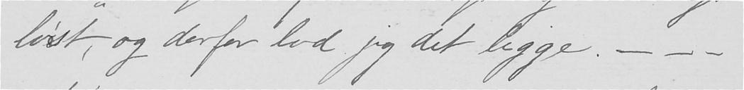løst, og derfor lod jeg det ligge. - - -
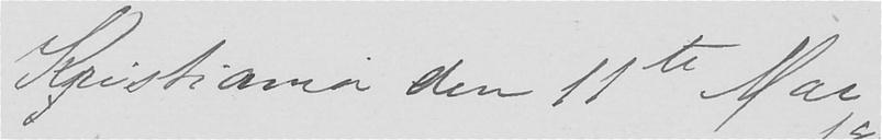Kristiania den 11te  Mai
